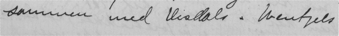sammen med Visdals. Wentzels
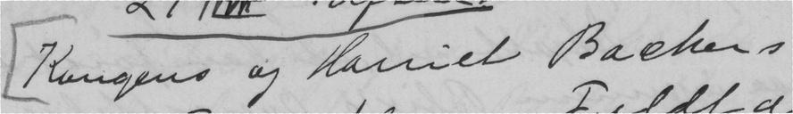Kongens og Harriet Backers
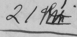21
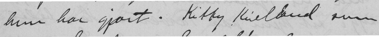hun har gjort. Kitty Kielland som
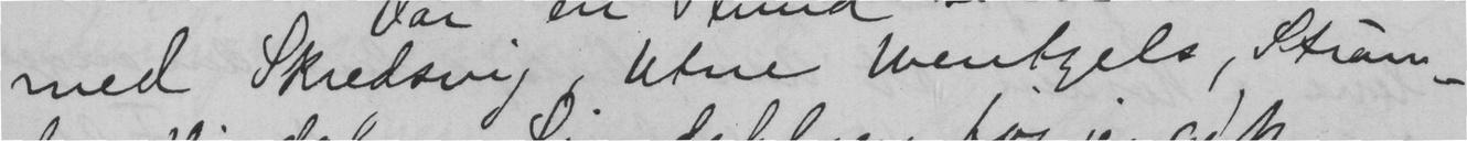med Skredsvig. Utne Wentzels, Strøm-
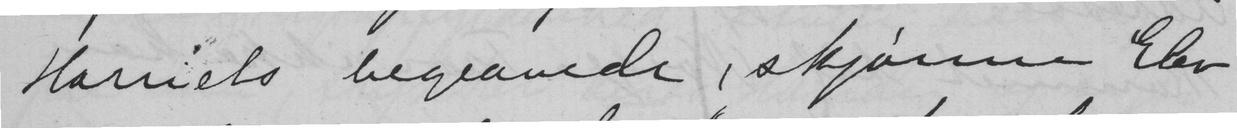Harriets begeavede, skjønne Elev
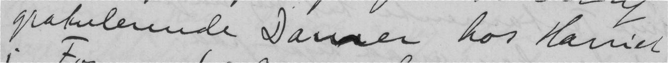gratulerende Damer hos Harriet
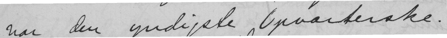var den yndigste Opværterske.
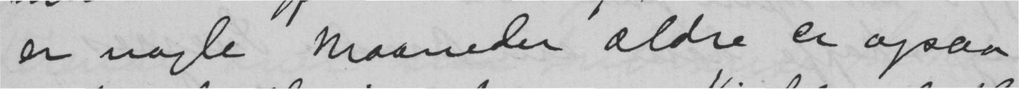er nogle maaneder ældre er ogsaa
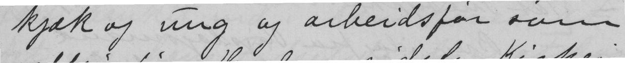kjæk og ung og arbeidsfør som
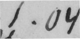1.04
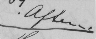. Aften.
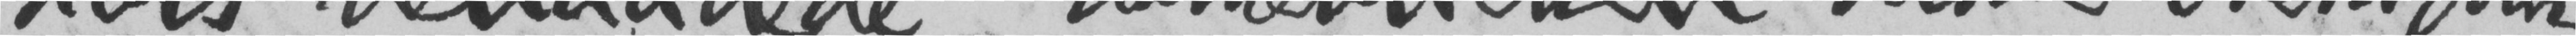kors benaadede. Udnævnelsen vakte øiensynlig
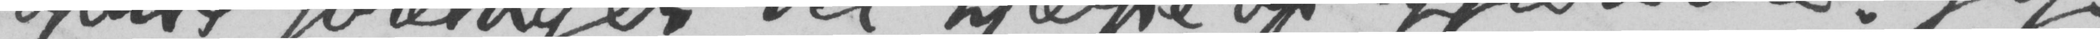april foretager, vil hjælpe op affærerne. Jeg
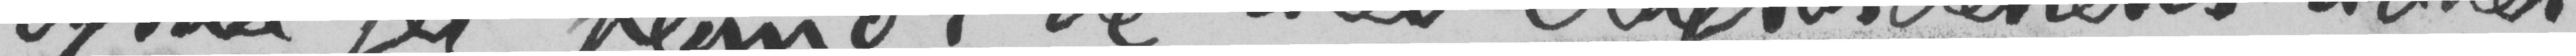ogsaa jeg blandt de med Olafsordenenes ridder-
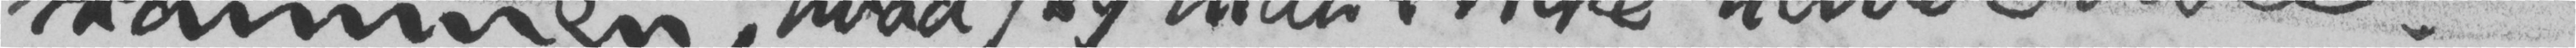skammen, hvad jeg hidtil ikke havde troet.
